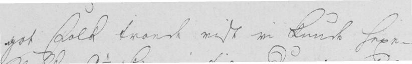got Folk troede vist vi kunde hexe -
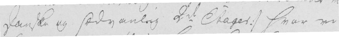Danske og sædvanlig 2de Etages :/ hvor vi
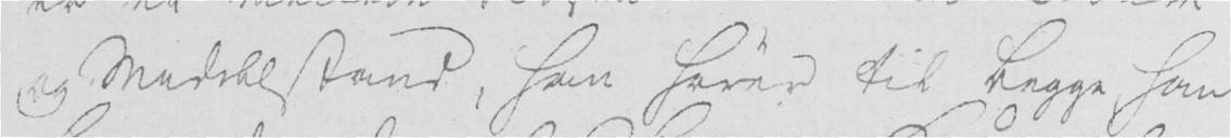og Middelstand, han hører til begge, han
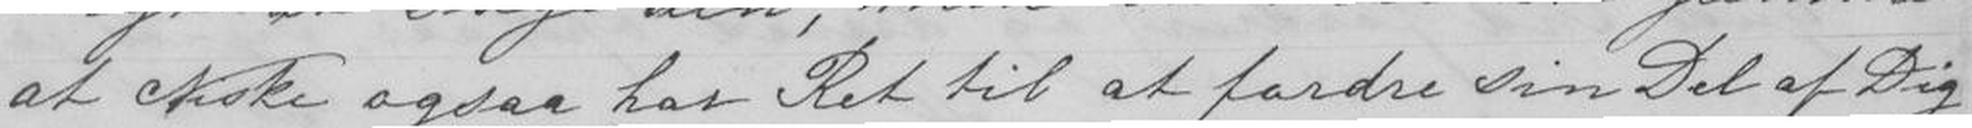at Niske ogsaa har Ret til at fordre sin Del af Dig
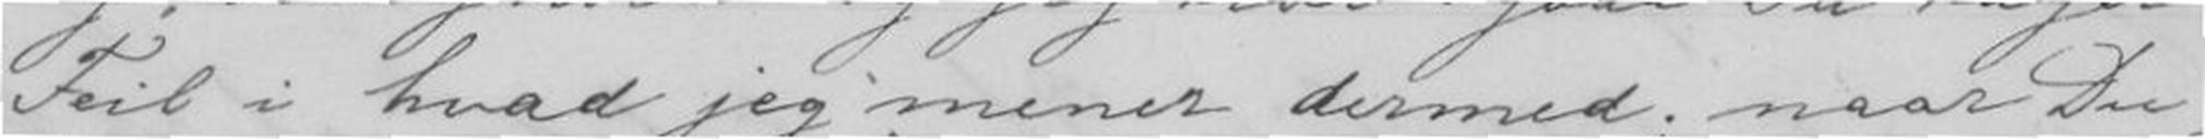Feil i hvad jeg mener dermed; naar Du
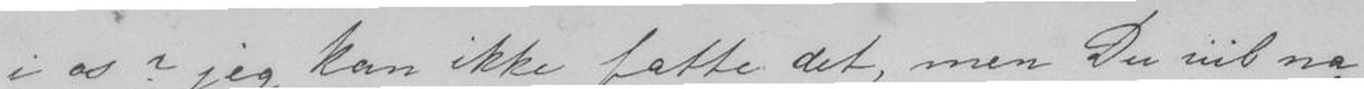i os? jeg kan ikke fatte det, men Du vil na
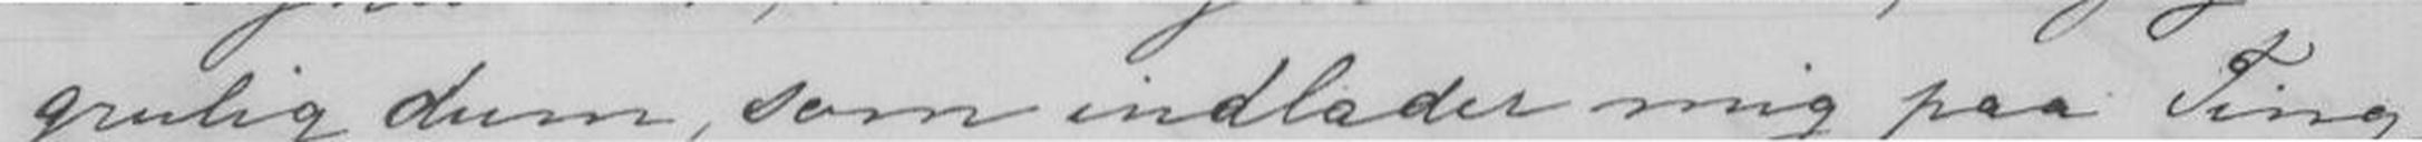grulig dum, som indlader mig paa Ting,
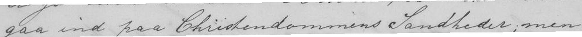gaa ind paa Christendommens Sandheder, men
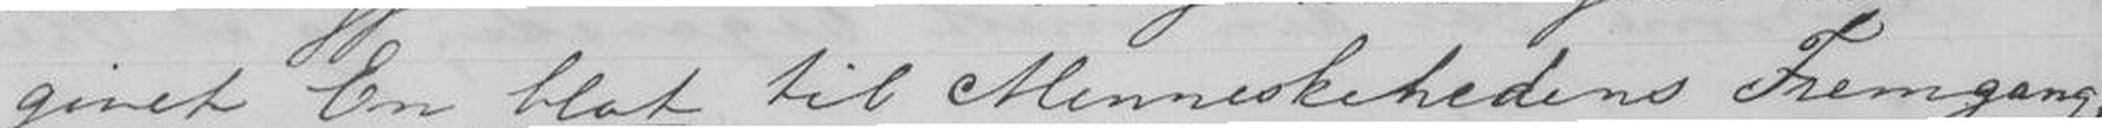givet En blot til Menneskehedens Fremgang,
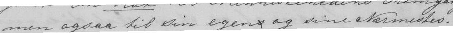men ogsaa til Din egen s og sine Nærmestes.
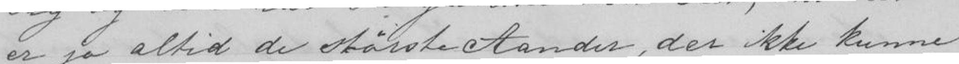er jo altid de største Aander, der ikke kunne
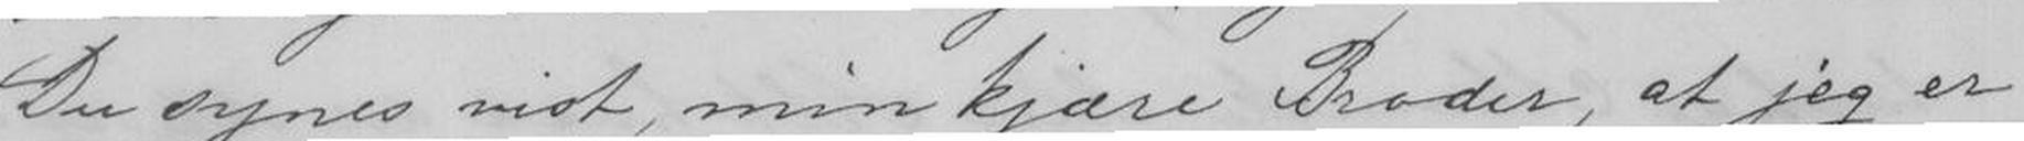Du synes vist, min kjære Broder, at jeg er
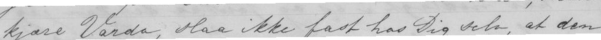kjære Vardo, slaa ikke fast hos Dig selv, at den
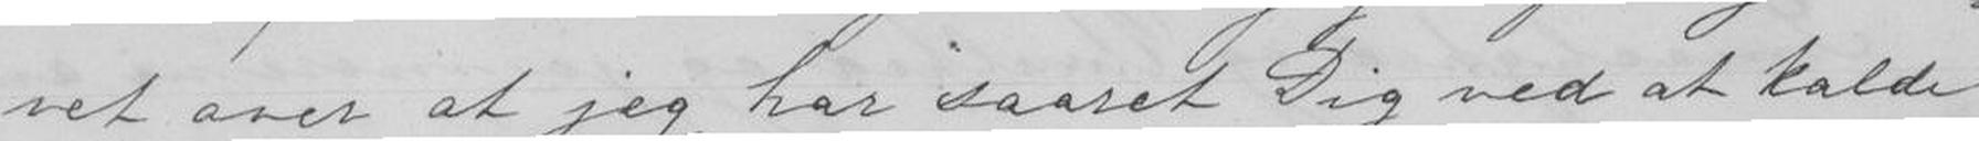vet over at jeg har saaret Dig ved at kalde
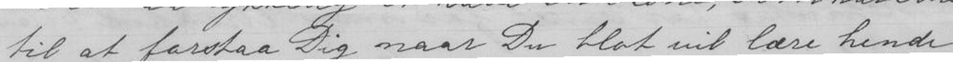til at forstaa Dig naar Du blot vil lære hende
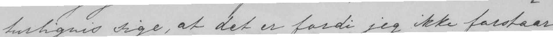turligvis sige, at det er fordi jeg ikke forstaar
